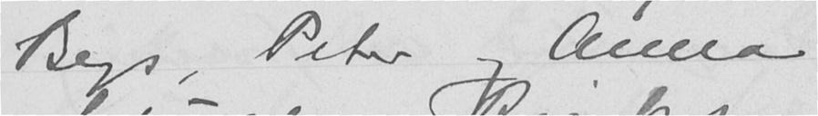Bergs, Peter og Anna
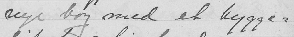nye bog med et hygge-
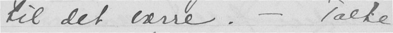til det værre. - Talte
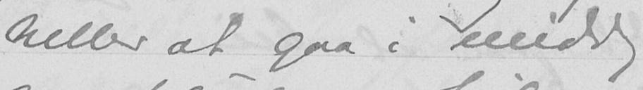heller at gaa i middag
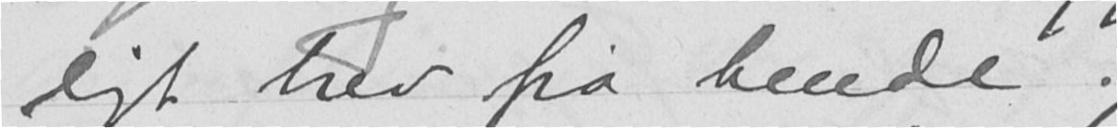ligt brev bra hende.
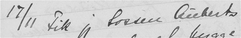17/11 Fik jeg Sossen Auberts
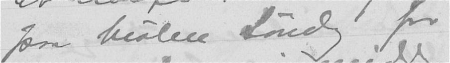paa Mølen Søndag for
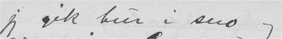jeg gik tur i sno og
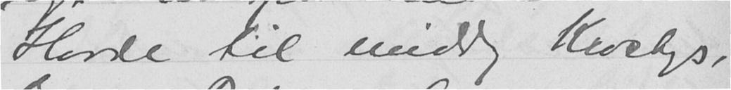Havde til middag Krosbys,
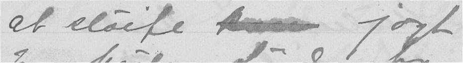at sløife kom jagt
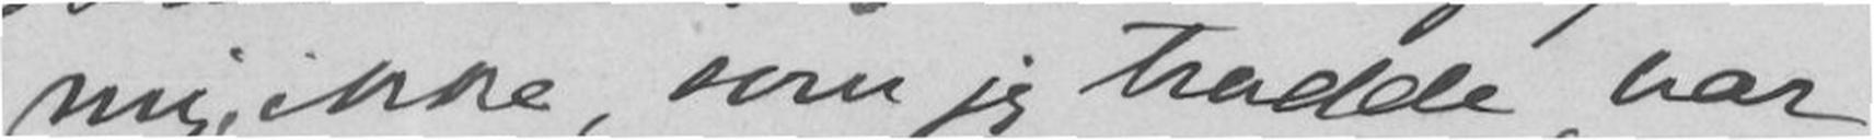mig, ikke, som jeg trodde, var
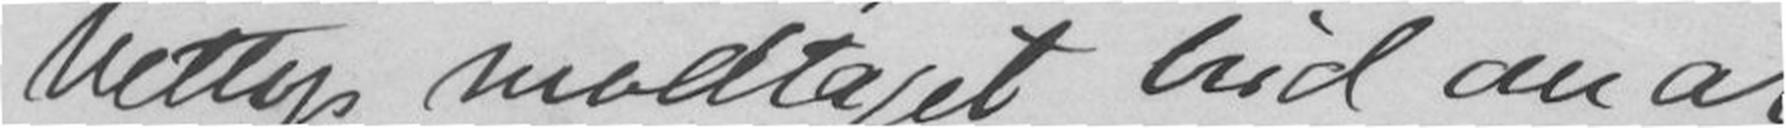Nettop modtaget bud om at
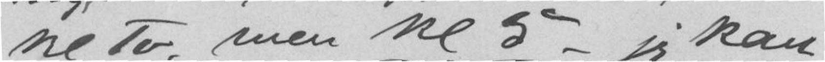til to, men kl 5 - jeg kan
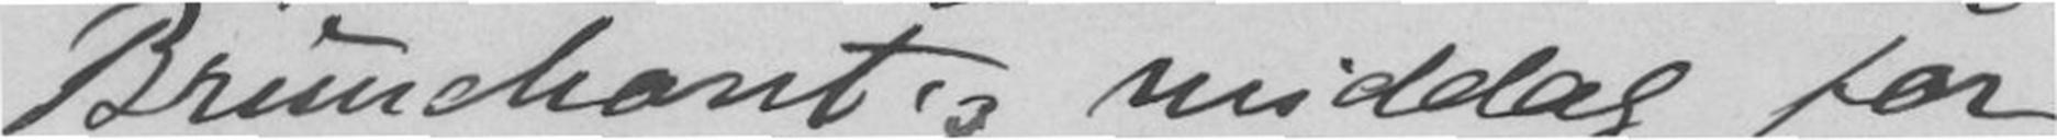Brunchant's middag for
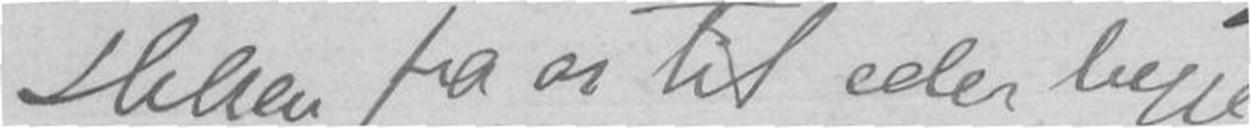Hilser fra os til eder begge!
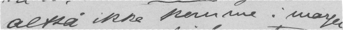altså ikke komme imorgen.
